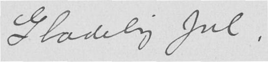Glædelig Jul,
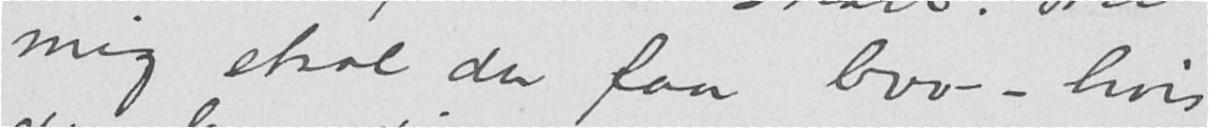mig skal du faa brev - hvis
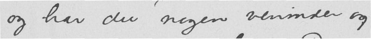og har du nogen veninder og
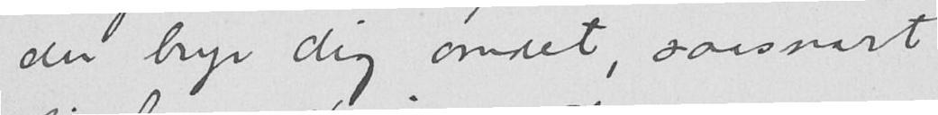du bryr dig om det, saasnart
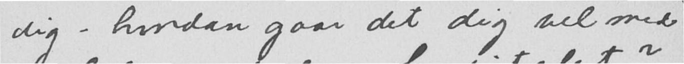dig - hvordan gaar det dig vel med
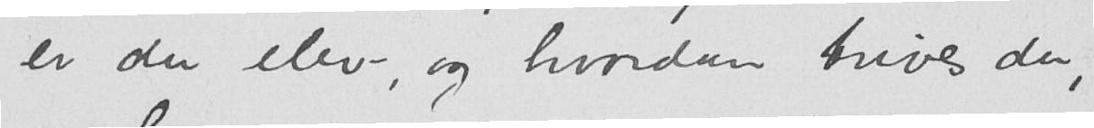er du elev, og hvordan trives du,
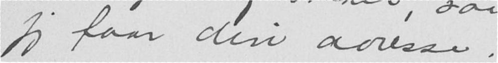jeg faar din adresse.
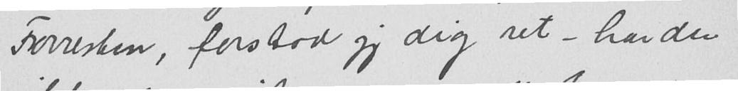Forresten, forstod jeg dig ret - har du
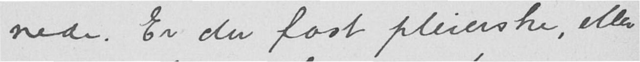nede. Er du fast pleierske, eller
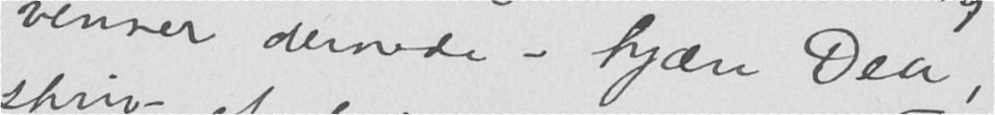venner dernede - kjære Dea,
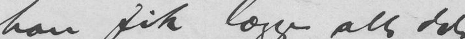han fik lægge alt det
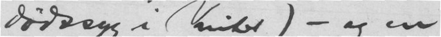Dødssyg i Vinter) - og en
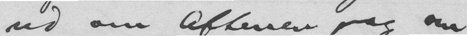ud om Aftenen og om
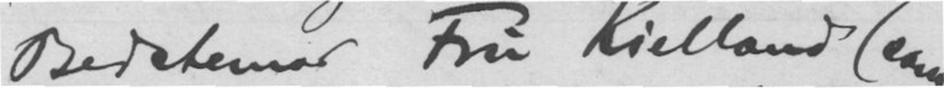Bedstemor Fru Kielland (som
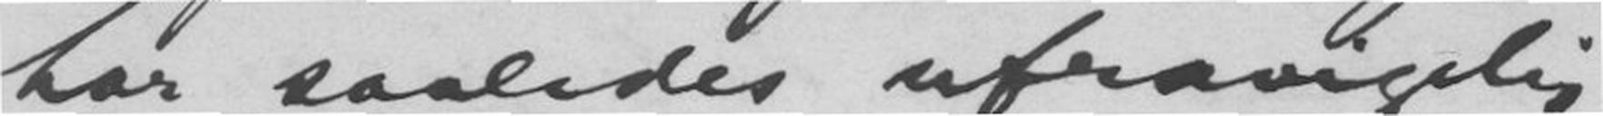har saaledes ufravigelig
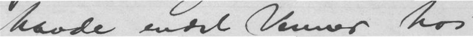havde endel Venner hos
